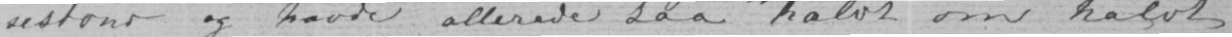sestour og havde allerede saa halvt om halvt
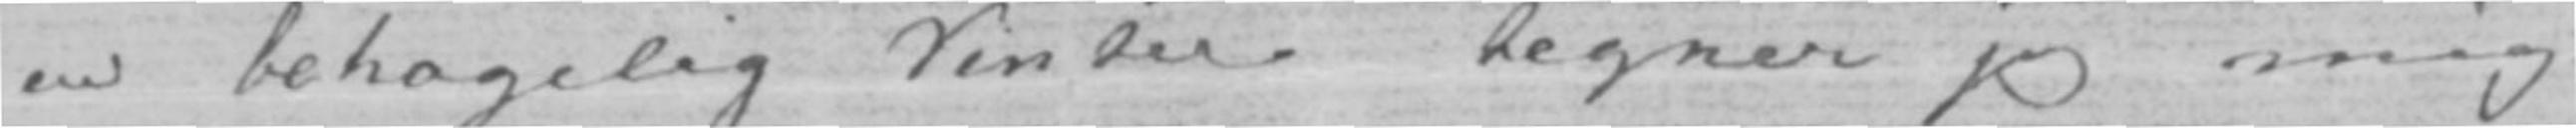en behagelig Vinter tegner jeg mig-
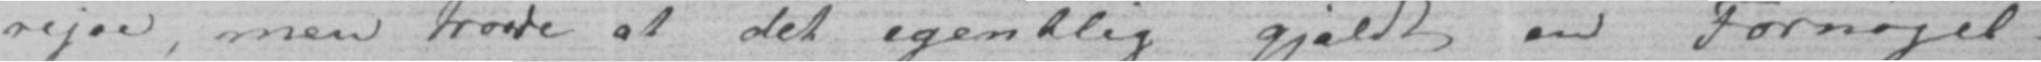rejse, men troede at det egentlig gjaldt en Fornøjel-
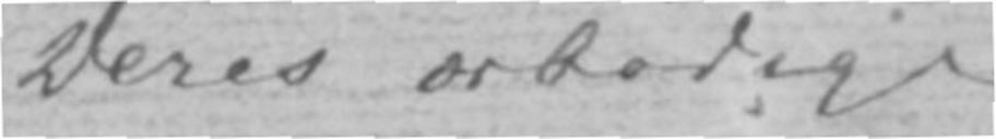Deres ærbødige
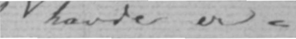havde er-
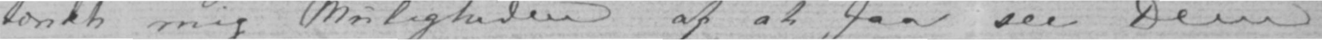tænkt mig Muligheden af at faa see Dem
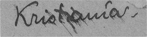Kristiania.
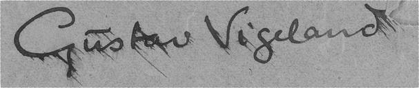Gustav Vigeland
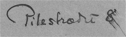Pilestrædet 8
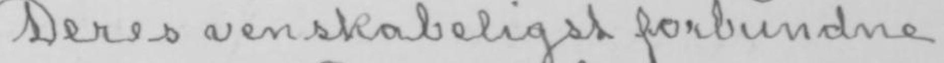Deres venskabeligst forbundne
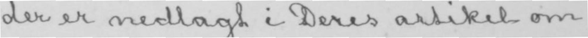der er nedlagt i Deres artikel om
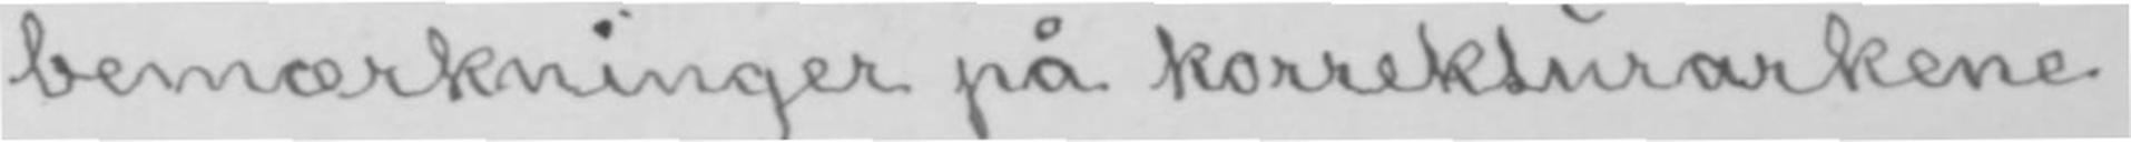bemærkninger på korrekturarkene.
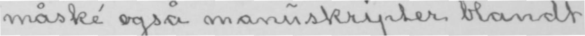måské også manuskripter blandt
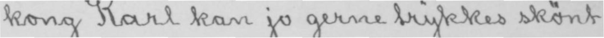kong Karl kan jo gerne trykkes skønt
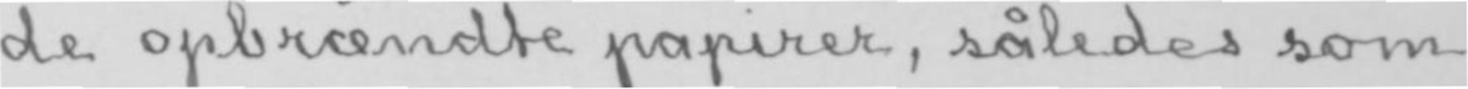de opbrændte papirer, således som
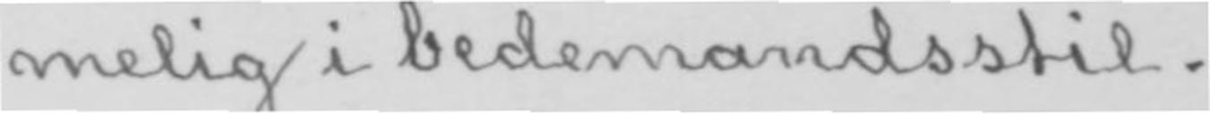melig i bedemandsstil.
